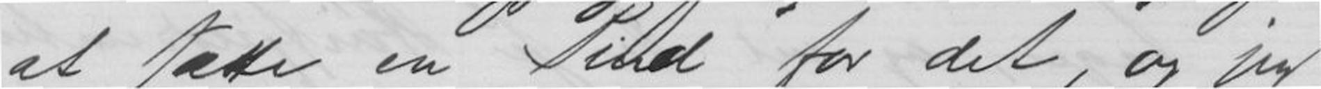at satte en Pind for det, og jeg
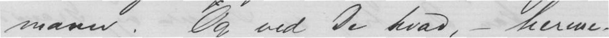mann. Og ved De hvad, - herme-
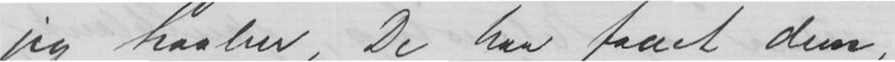jeg haaber, De har faaet dem, -
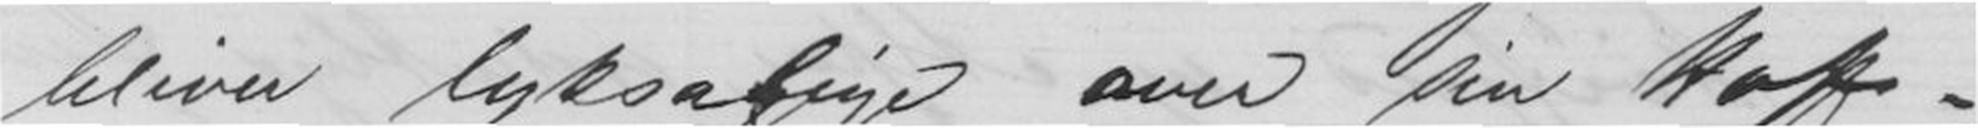bliver lyksalige over sin Hoff-
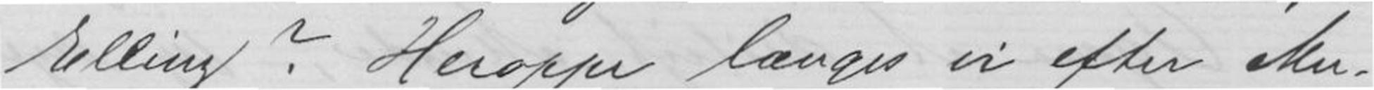Elling? Heroppe længes vi efter Mu-
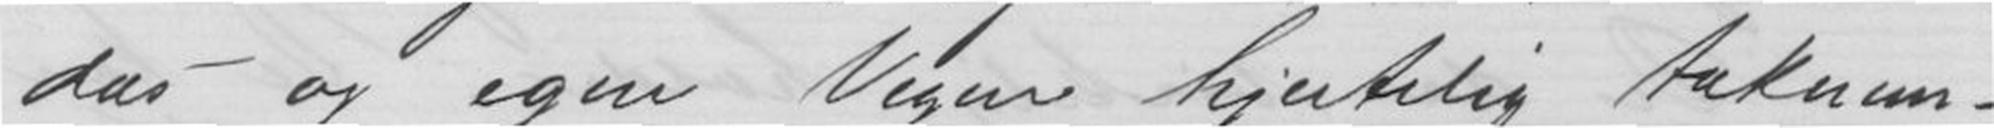das og egne Vegne hjertelig taknem-
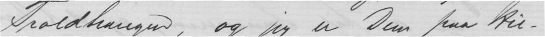Troldhaugen, og jeg er Dem paa Hil-
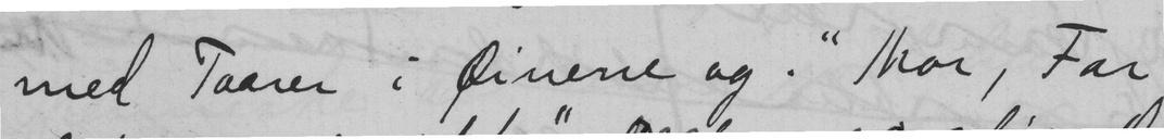med Taarer i Øinene og. "Mor, Far
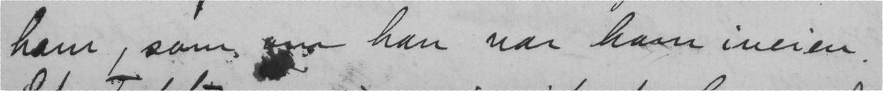ham, som om han var ham i veien.
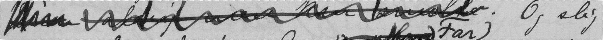. Og slig
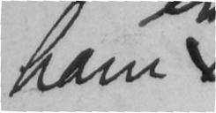ham
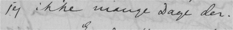jeg ikke mange Dage der.
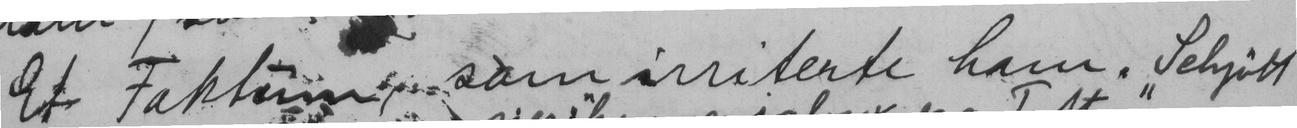Et Faktum som irriterte ham. Schjøtt
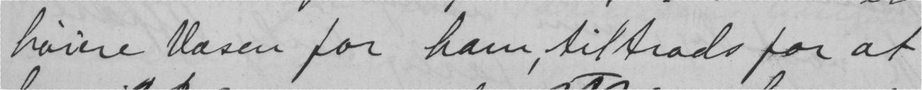høiere væsen for ham, tiltrods for at
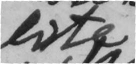lite
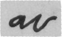av
